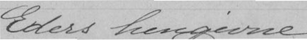Edres hengivne
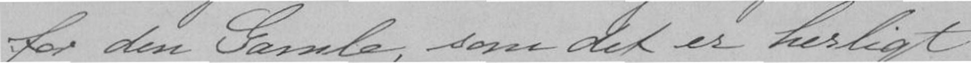for den Gamle, som det er herligt
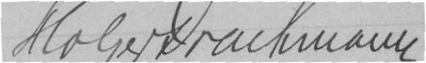Holger Drachmann
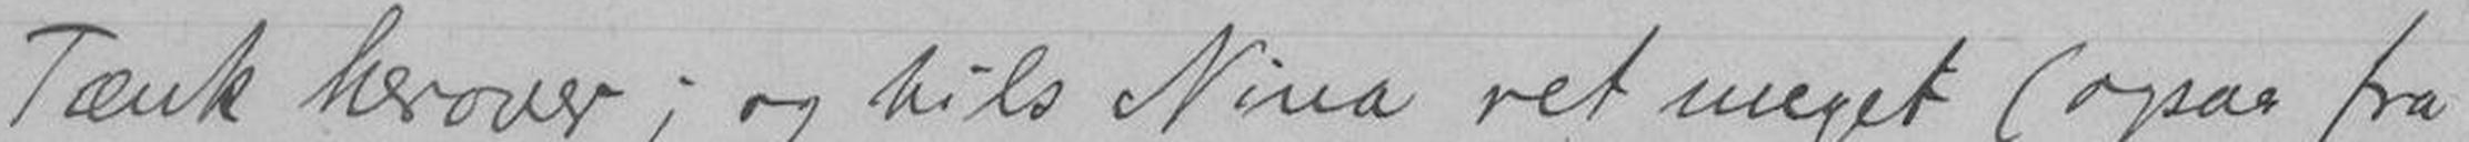Tænk herover; og hils Nina ret meget (ogsaa fra
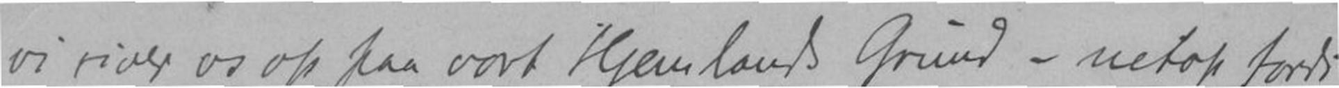vi river os op fra vort Hjemlands Grund - netop fordi
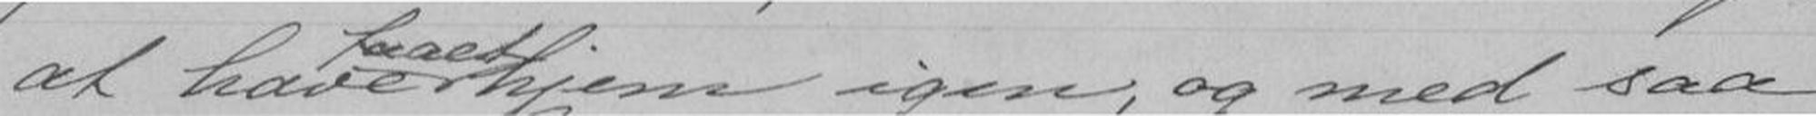at have hjem igen, og med saa
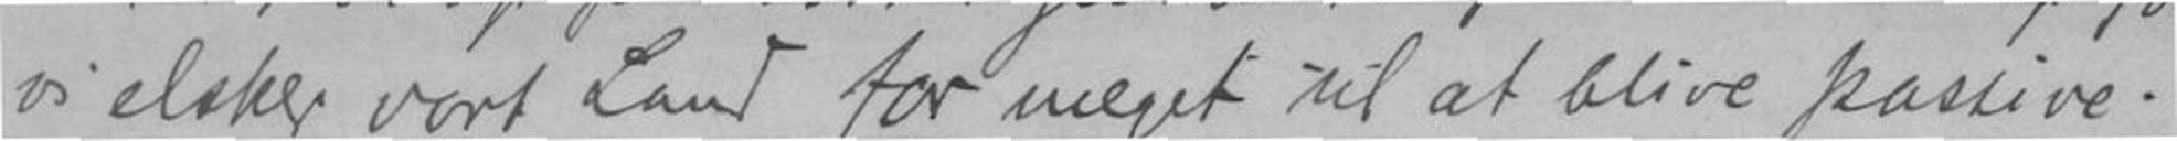vi elsker vort Land for meget til at blive passive.
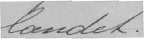landet.
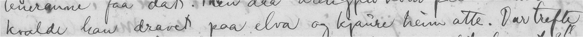kvalde han dravet paa elva og kjaure heim atte. Dar trefte
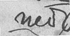ned
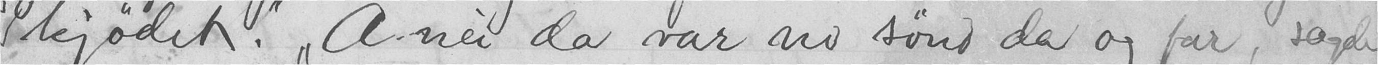skjødet." "Anei da var no sønd da og for, sagde
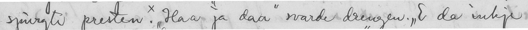spurgte presten. "Haa ja daa" svarde drengen. "E da inkje
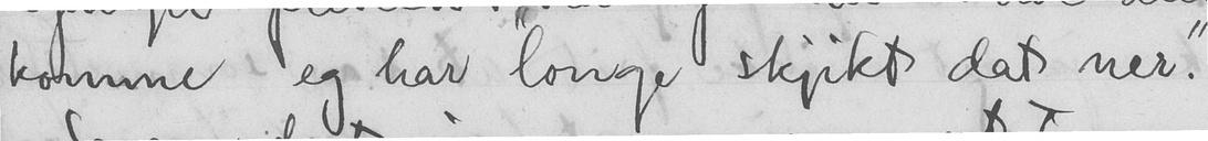komme eg har longe skjikt dat ner."
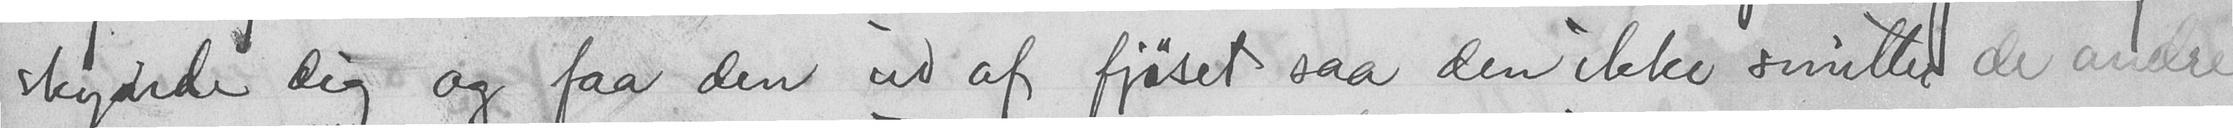skynde dig og faa den ud af fjøret saa den ikke smitter de andre
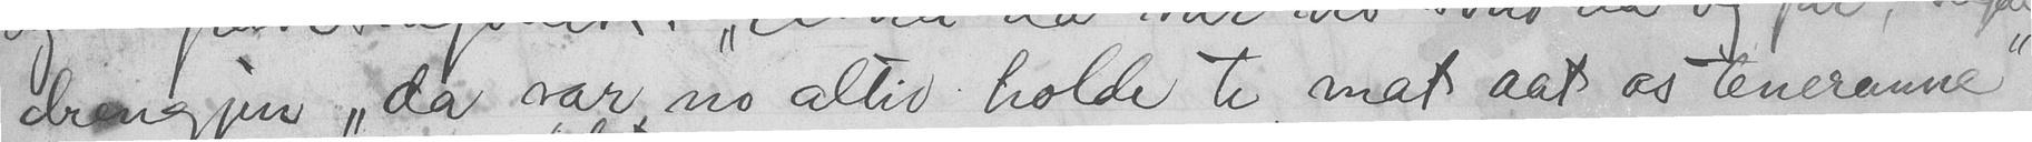drengjen "da var no altid holde te mat aat os teneranne"
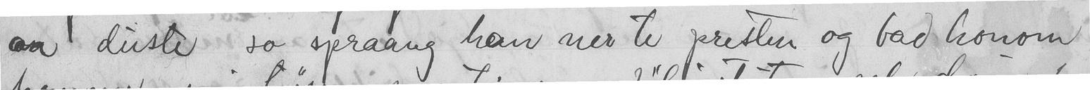aa duste so spraang han ner te presten og bad honom
